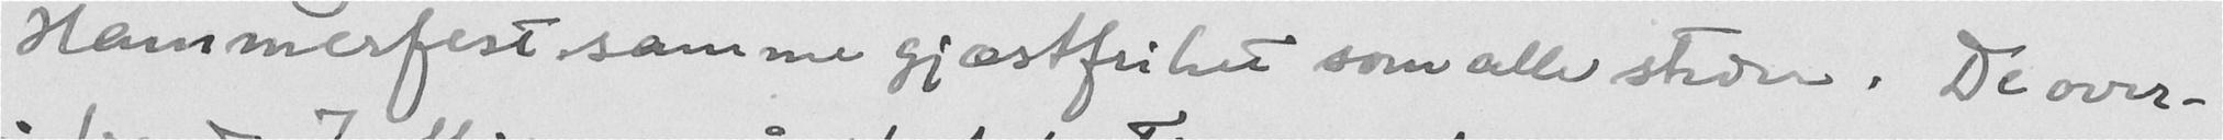Hammerfest samme gjæstfrihet som alle steder. De over-
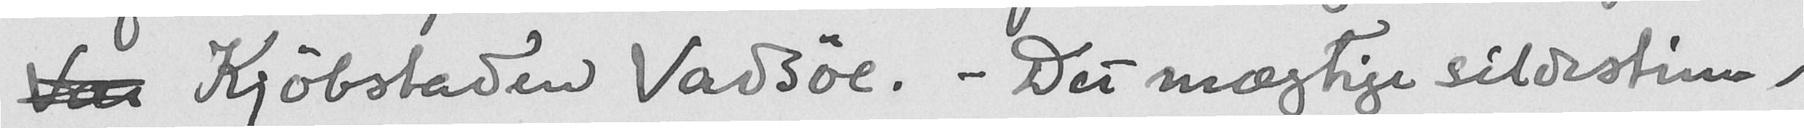Va Kjøbstaden Vadsøe. - Det mægtige sildestim,
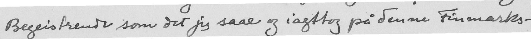Begeistrende som det jeg saae og iagttog på denne Finmarks-
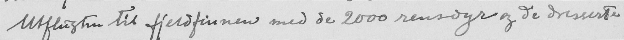Utflugten til fjeldfinnen med de 2000 rensdyr og de dresserte
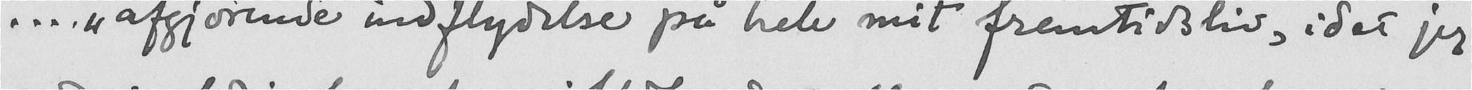... "afgjørende indflydelse på hele mit fremtidsliv, idet jeg
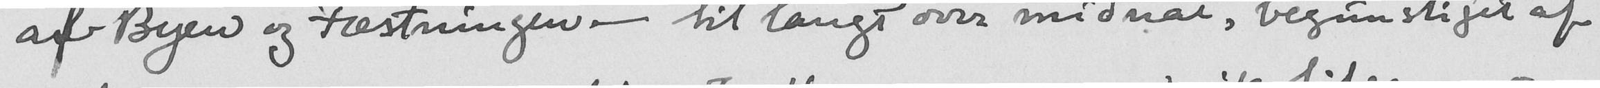af Byen og Fæstningen - til langt over midnat, begunstiget af
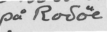på Rodøe
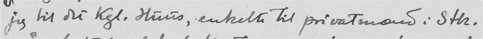jeg til det Kgl. Huus, enkelte til privatmænd i Sth.
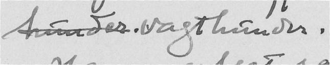hunder vagthunder.
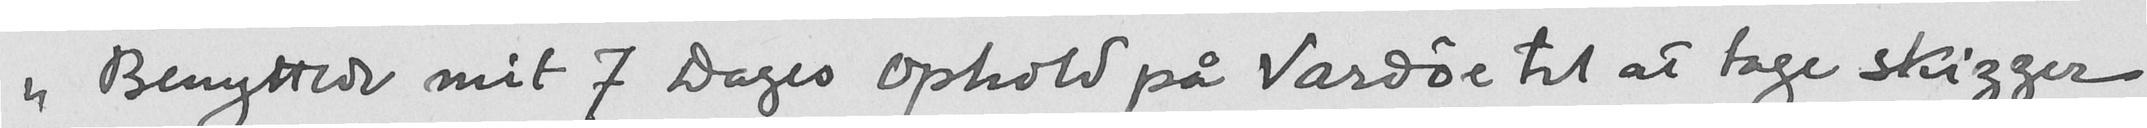"Benyttede mit 7 Dages ophold på Vardøe til at tage skizzer
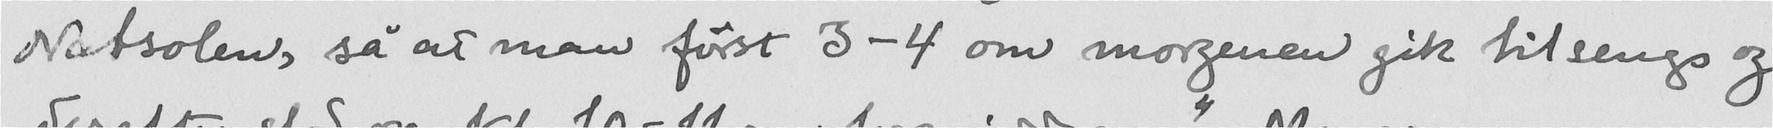Natsolen, så at man først 3 - 4 om morgenen gik tilsengs og
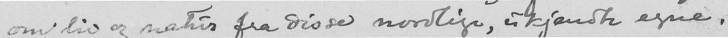om liv og natur fra disse nordlige, ukjendte egne.
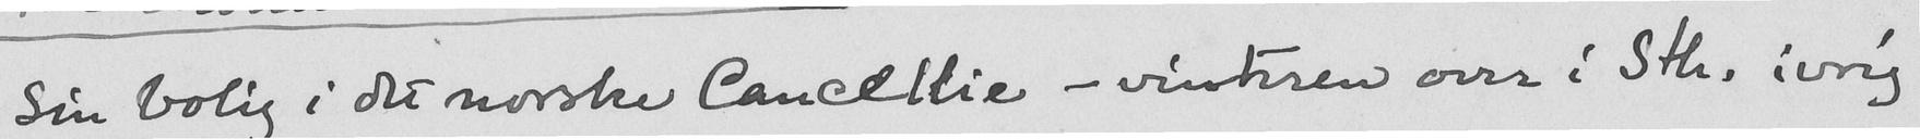Sin bolig i det norske Cancellie - vinteren over i Sth. ivrig
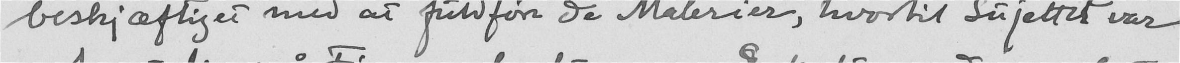beskjæftiget med at fuldføre de Malerier, hvortil sujettet var
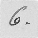6.
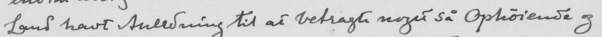Land havt Anledning til at betragte noget så Ophøiende og
